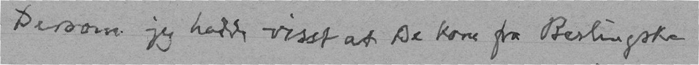Dersom jeg hadde visst at De kom fra Berlingske
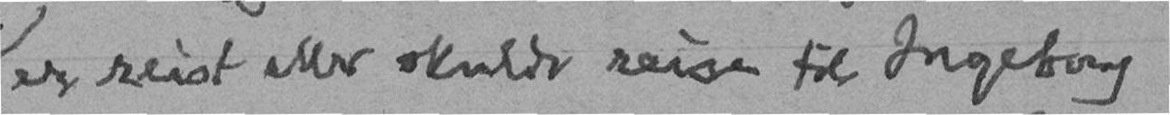er reist eller skulde reise til Ingeborg
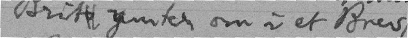Britt ymter om i et Brev
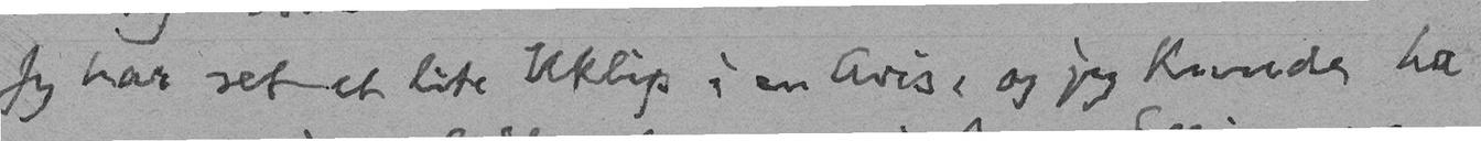Jeg har set et lite Uklip i en Avis, og jeg kunde ha
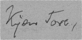Kjære Tore,
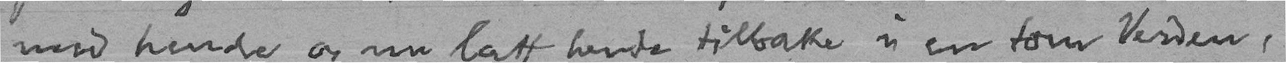med hende og nu latt hende tilbake i en tom Verden,
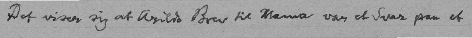Det viser sig at Arilds Brev til Mama var et Svar paa et
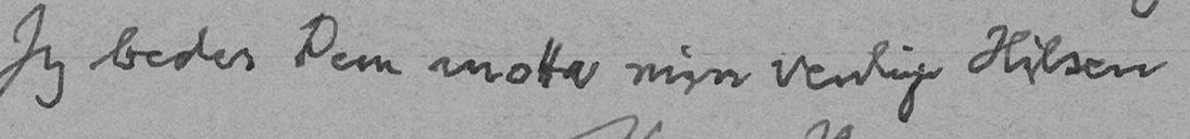Jeg beder Dem motta min venlige Hilsen
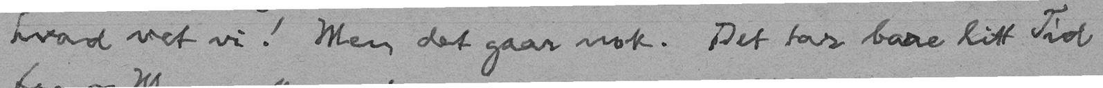hvad vet vi! Men det gaar nok. Det tar bare litt Tid
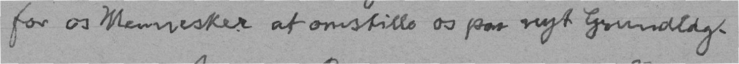for os Mennesker at omstille os paa nyt Grundlag.
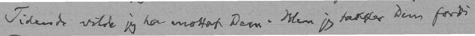Tidende vilde jeg ha mottat Dem. Men jeg takker Dem fordi
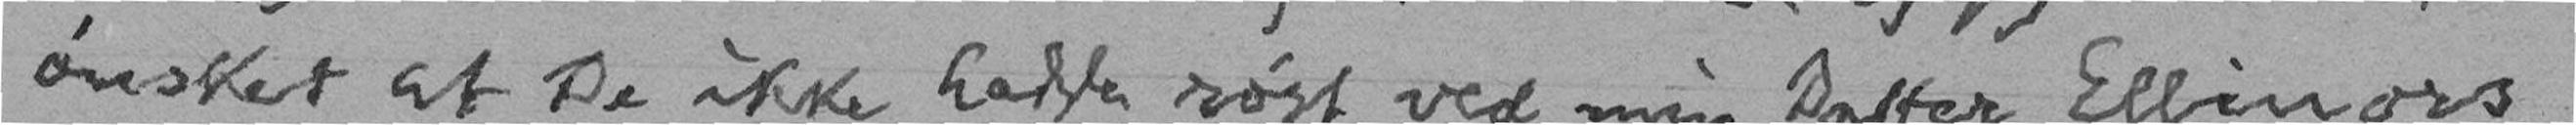ønsket at De ikke hadde rørt ved min Datter Ellinors
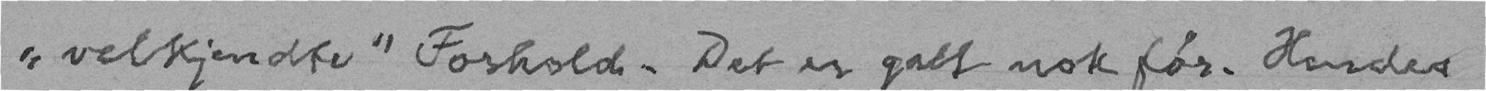"velkjendte" Forhold. Det er galt nok før. Hendes
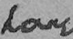har
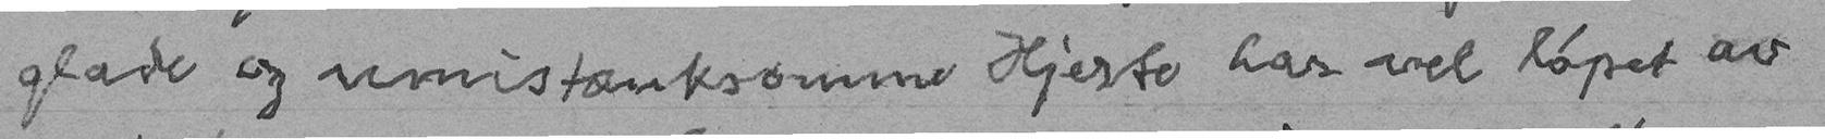glade og umistænksomme Hjerte har vel løpet av
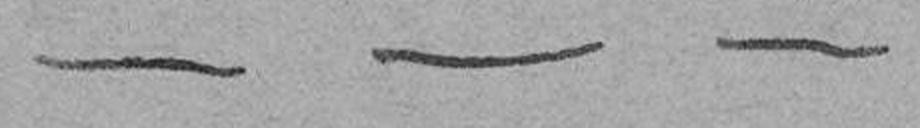- - -
